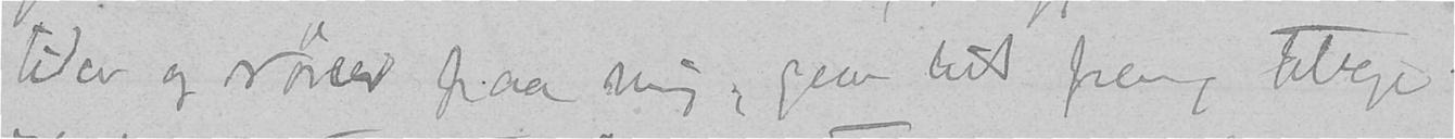tider og rører paa mig, gaa lidt frem og tilbage.
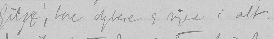Gilje, bare dybere og rigere i alt.
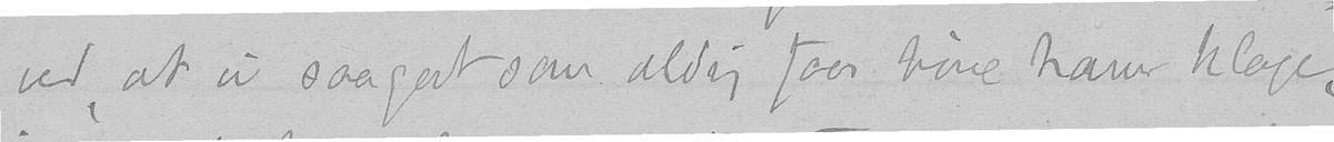ved, at vi saagodt som aldrig faar høre ham klage,
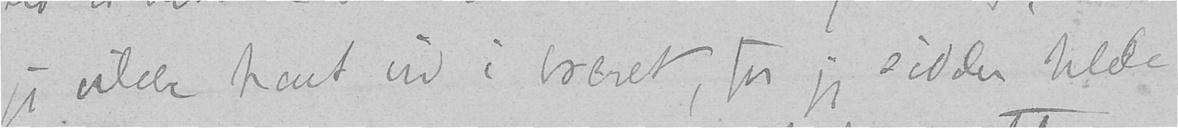jeg vilde havt ud i brevet, for jeg sidder hele
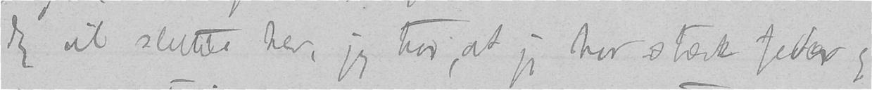Jeg vil slutte her, jeg tror, at jeg har stærk feber og
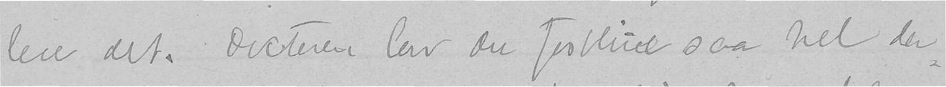leve det. Docteren lar du forblive saa hel der-
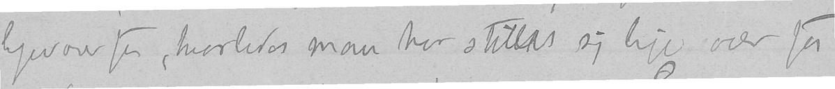ligevoverfor, hvorledes man har stilled sig lige overfor
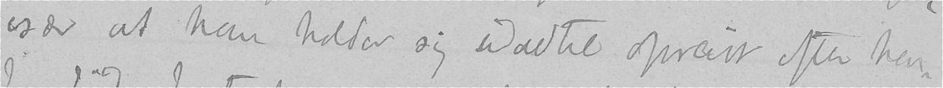især at han holder sig udadte opreist efter hen-
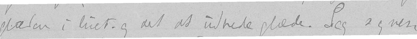glæden i livet og det at udbrede glæde. Jeg synes,
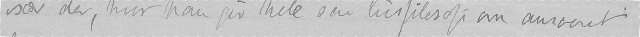især der, hvor han gir hele sin livsfilosofi om ansvaret
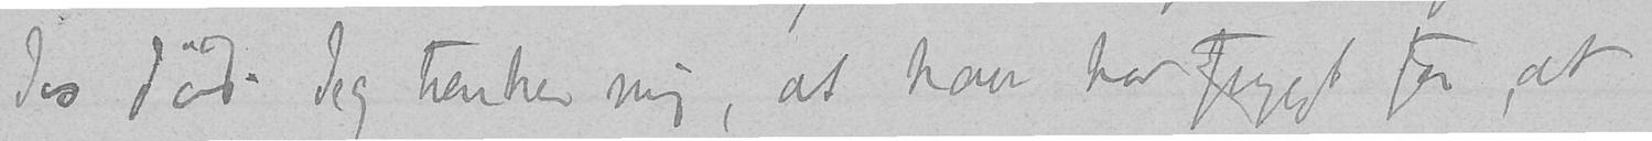des død. Jeg tænker mig, at han har frygt for, at
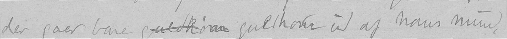der gaar bare guldkørn guldkorn ud af hans mund,
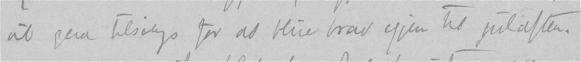vil gaa tilsengs for at blive brav igjen tid julaften.
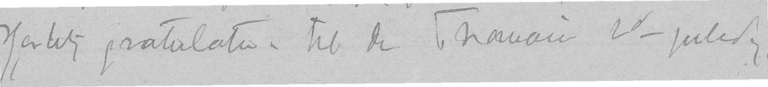Hjertelig gratulation til de Thomasine 2d - juledg,
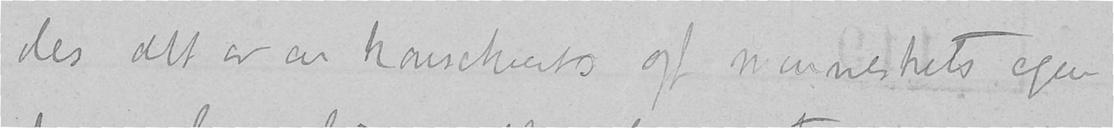des alt er en konsekvents af menneskets egen
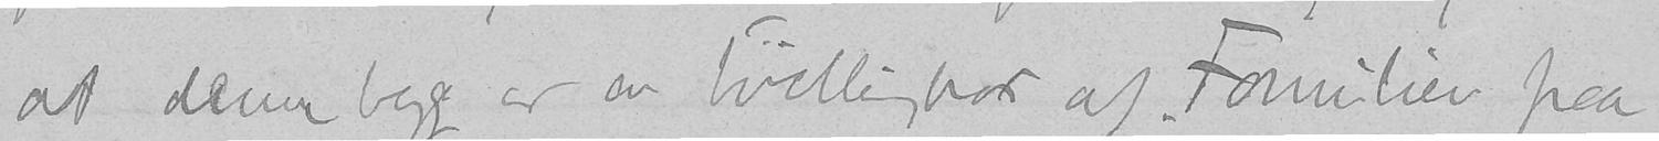at denne bog er en tvillingbror af Familien paa
